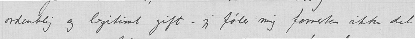ordentlig og legitimt gift – jeg føler mig forresten ikke det
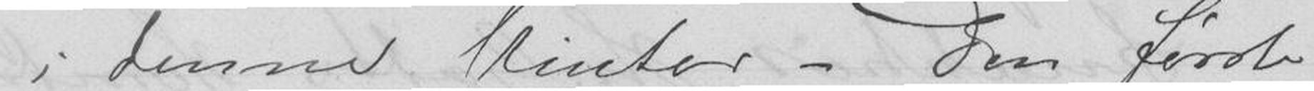i denne Vinter - Den første
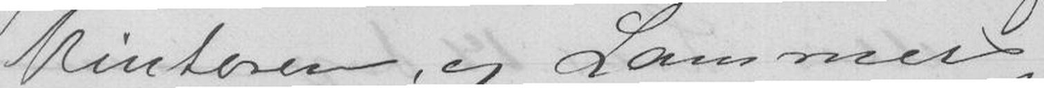Vinteren, og Lammers
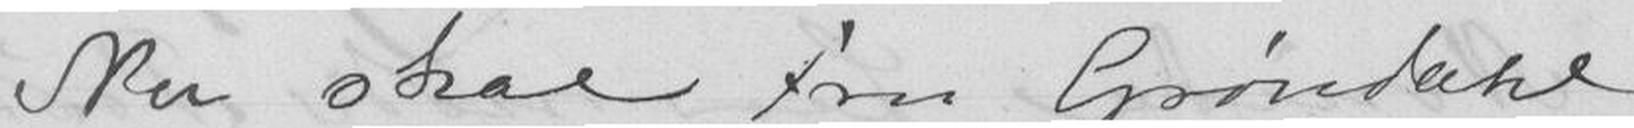Nu skal Fru Grøndahl
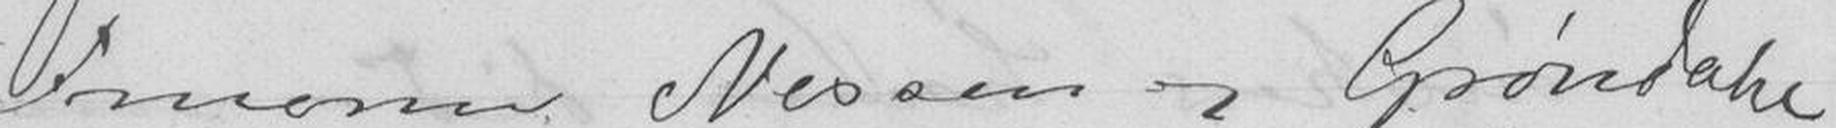Fruerne Nissen og Grøndahl
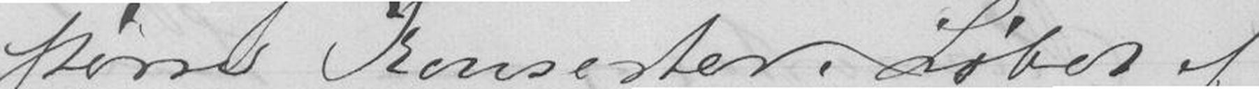større Konserter. Løbet af
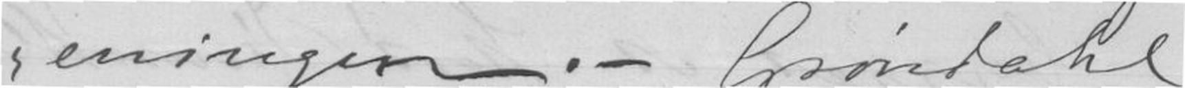eningen. - Grøndahl
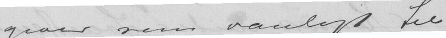gaar som vanligt be
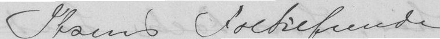Ibsens Folkefiende
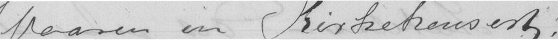giver en Kirkekonsert.
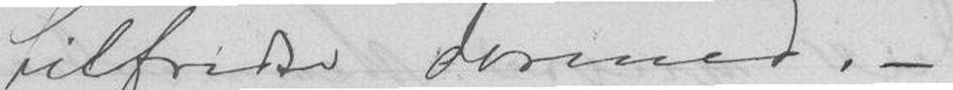tilfreds dermed. -
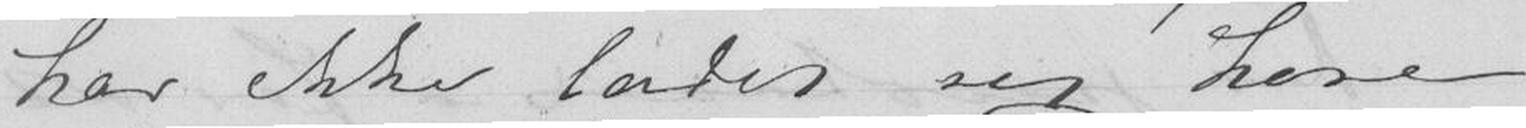har ikke ladet sig høre
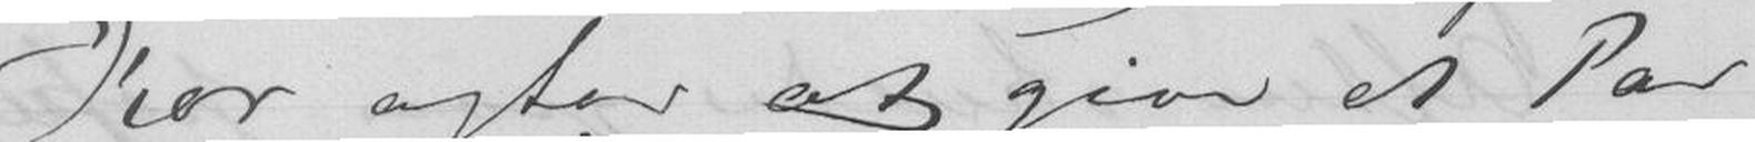Kor agter at give et Par
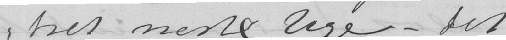tret neste Uge - det
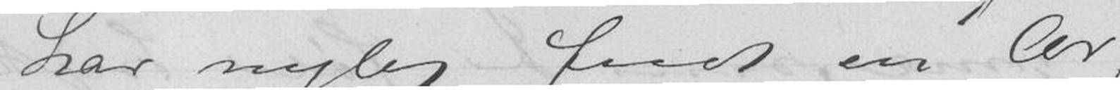har nylig faaet en Ar-
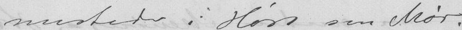mistede i Høst sin Mór.
